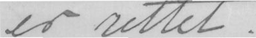er rettet.
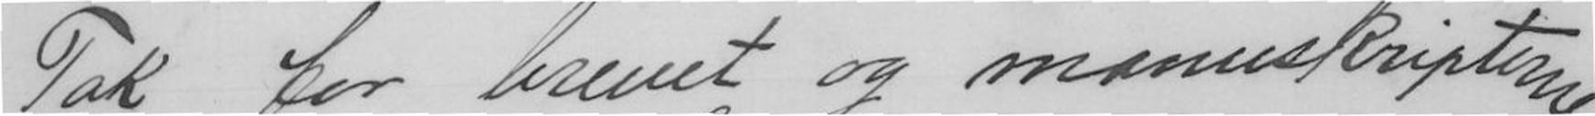Tak for brevet og manuskripterne.
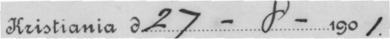Kristiania d. 27-8- 1901.
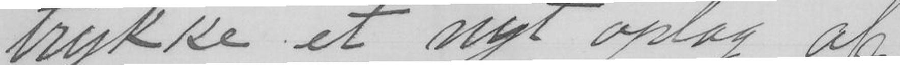trykke et nyt oplag af
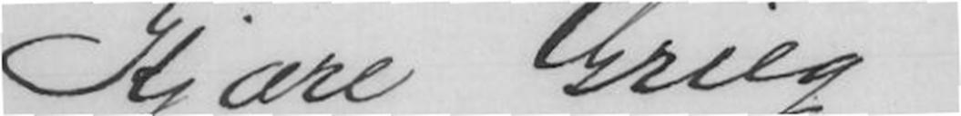Kjære Grieg
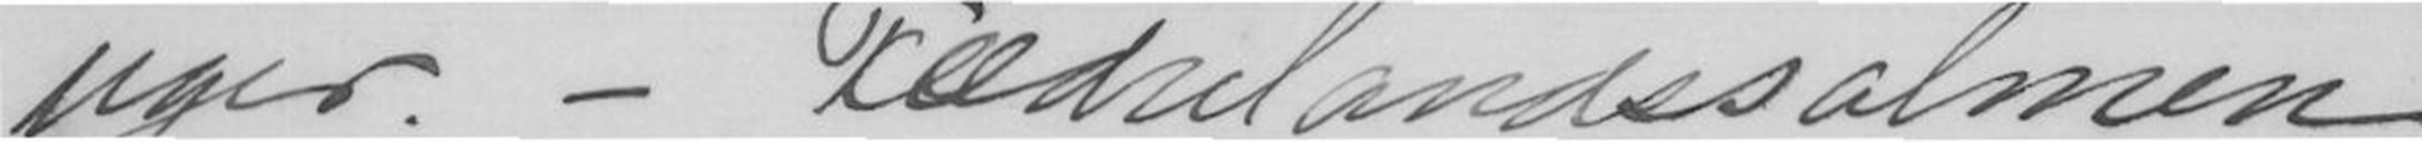uger. - Fædrelandssalmen
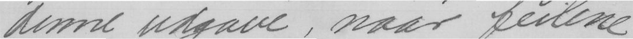denne udgave, naar feilene
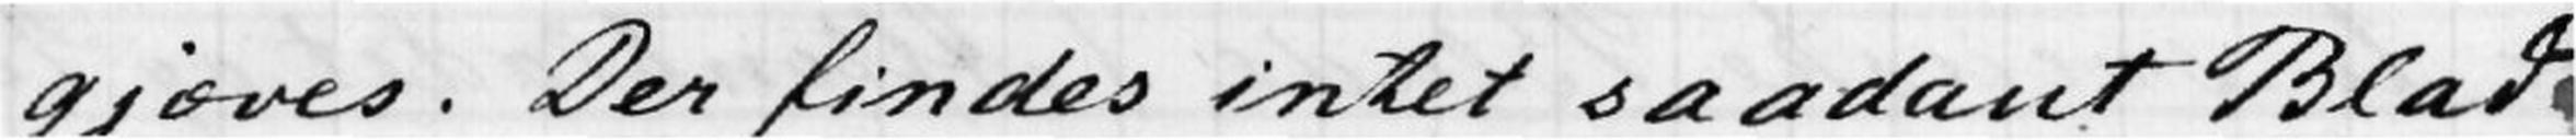gjøres. Der findes intet saadant Blad
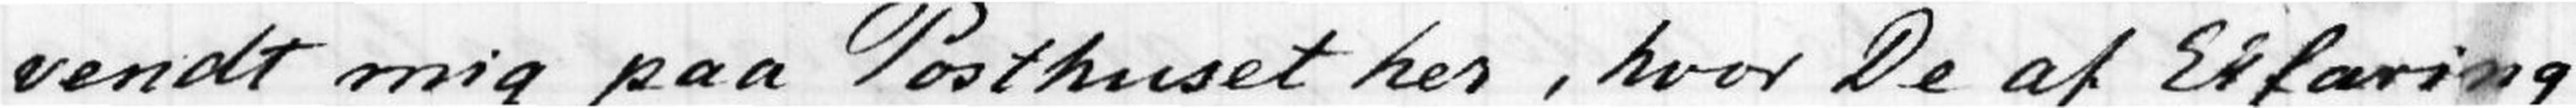vendt mig paa Posthuset her, hvor De af Erfaring
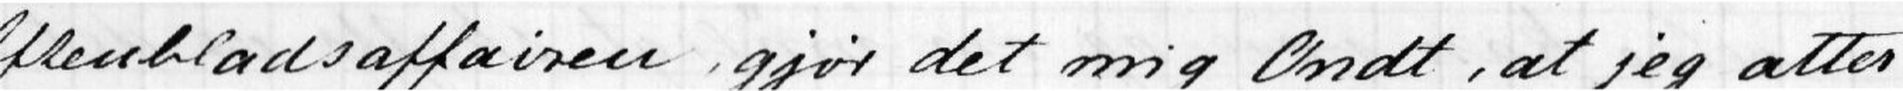Aftenbledsaffairen gjør det mig Ondt, at jeg atter
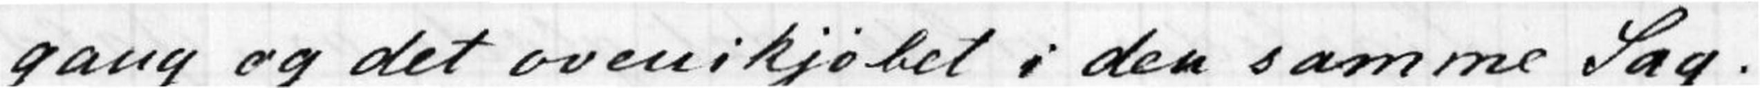gang og det ovenikjøbet i den samme Sag.
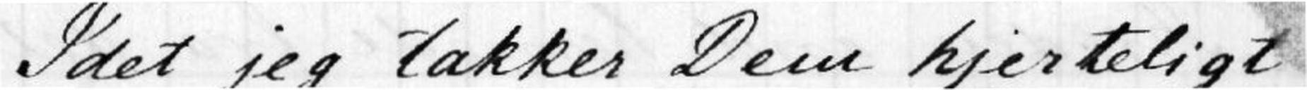Idet jeg takker Dem hjerteligt
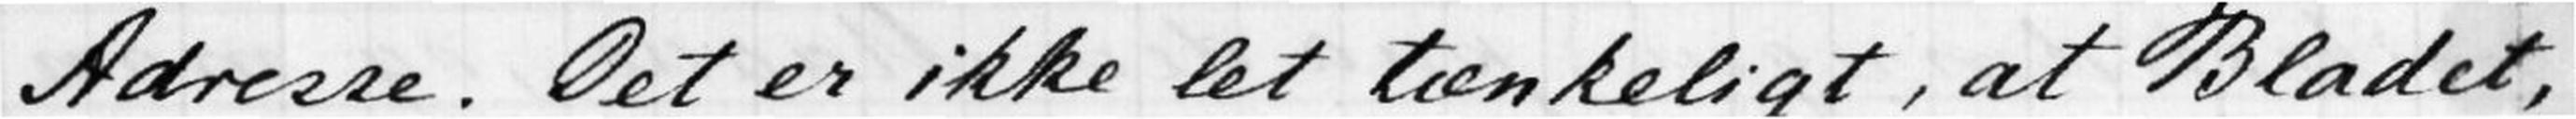Adresse. Det er ikke let tænkeligt, at Bladet,
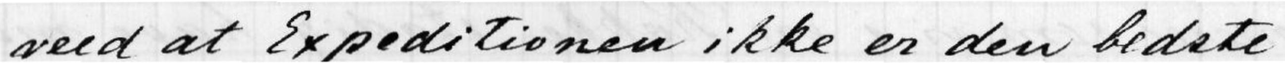veed at Expeditionen ikke er den bedste,
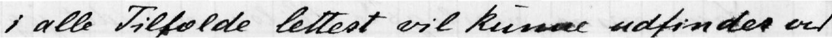i alle Tilfælde lettest vil kunne udfindes ved
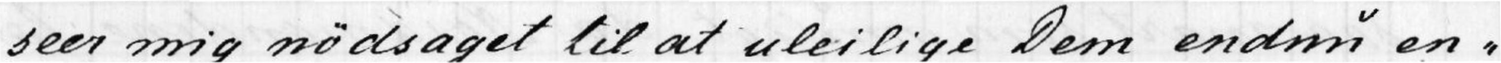seer mig nødsaget til at uleilige Dem endnu en-
